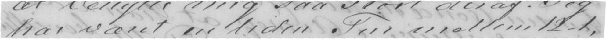har været en liden Tur mellem 12-1,
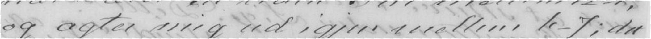og agter mig ud igjen mellem 6-7; det
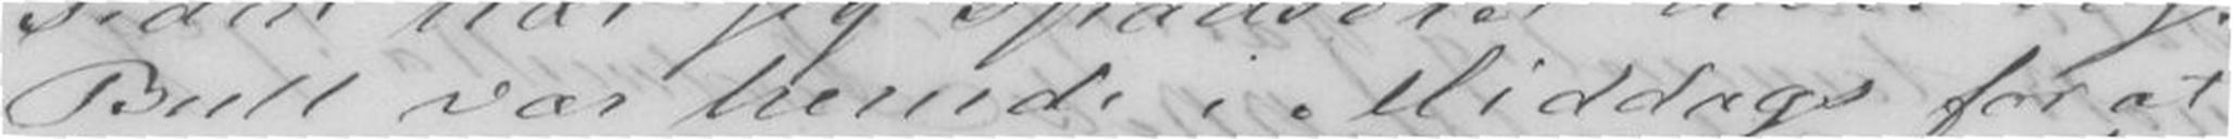Bull var herude i Middags for at
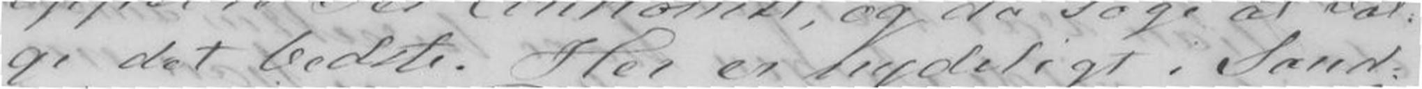ga det bedste. Her er nydeligt i Sand-
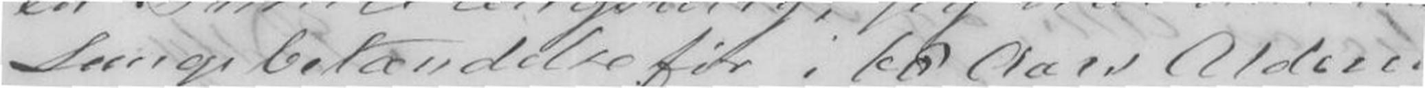Lungebetændelse før i 60 Aars Aldere
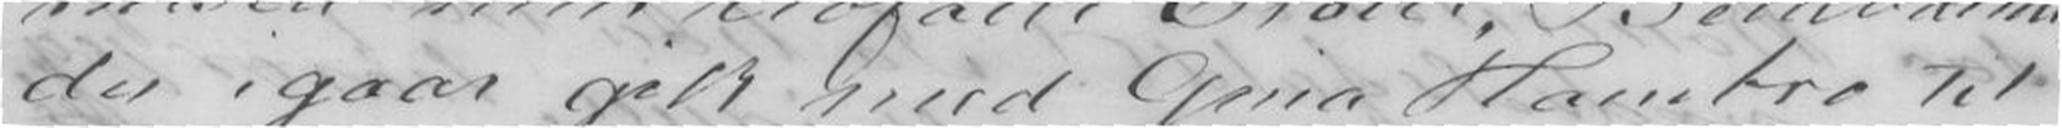der igaar gik med Gine Hambro til
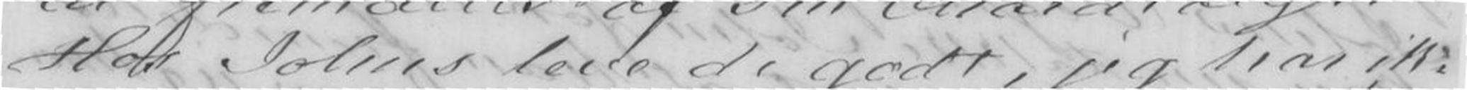Hos Johns leve de godt, jeg har ik-
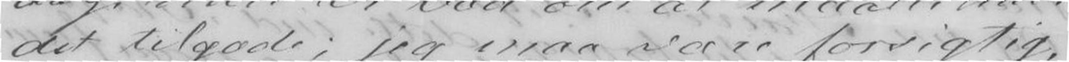det tilgode; jeg maa være forsigtig,
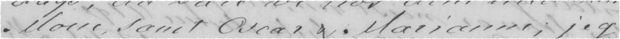Mone samt Oscar og Marianne; jeg
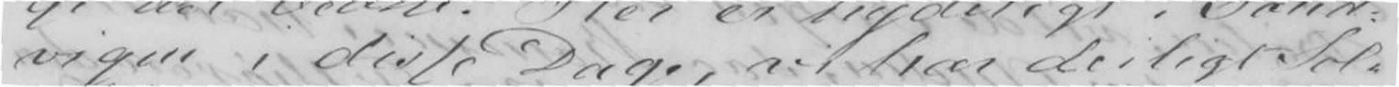vigen i disse Dage, vi har deiligt Sol-
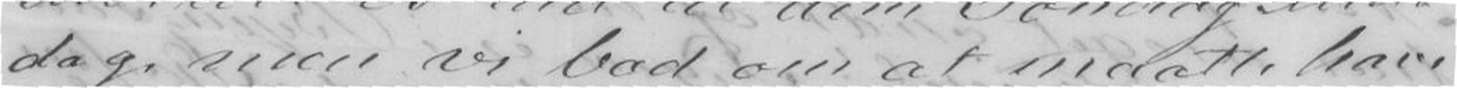dag, men vi bad om at maatte have
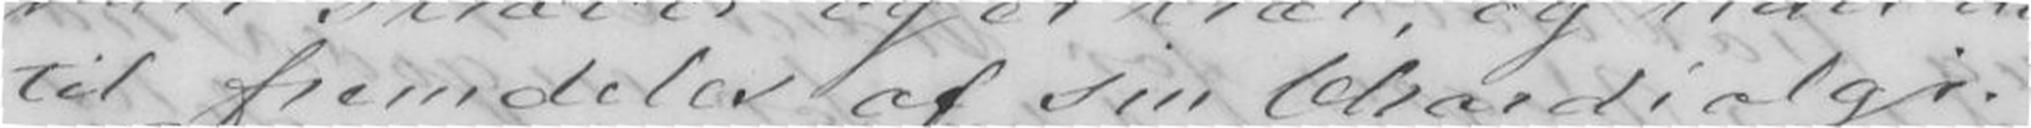til fremdeles af sin Chardialgi.
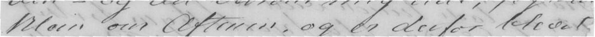klein om Aftenen, og er derfor blevet
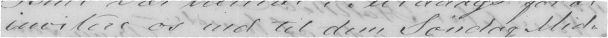invitere os ind til dem Søndag Mid-
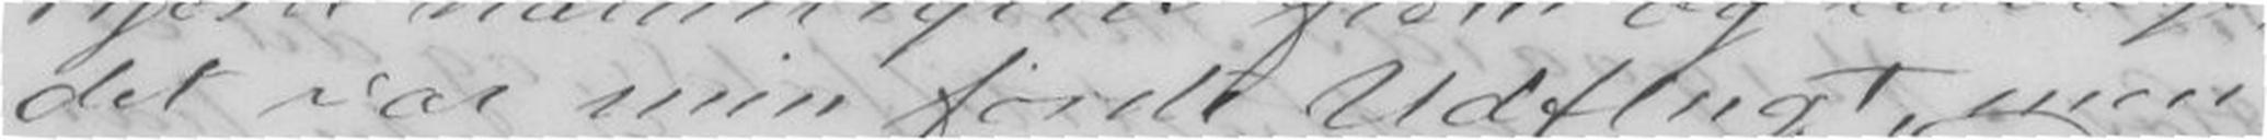det var min første Udflugt, men
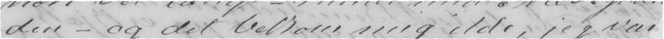den - og det bekom mig ilde, jeg var
